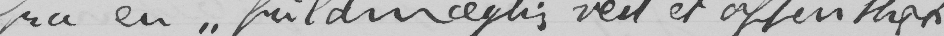fra en «fuldmægtig ved et offentligt
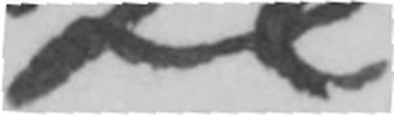se
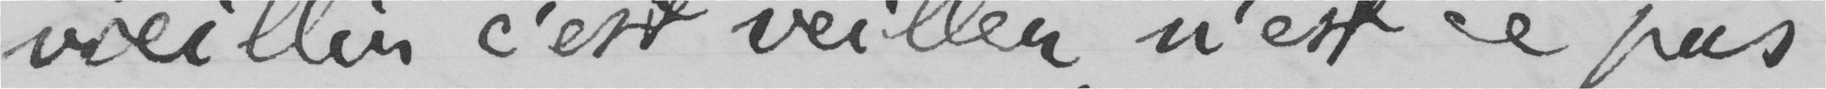vicillir c’est veiller, n’est ce pas?
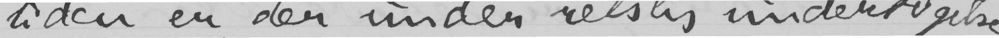tiden er der under retslig undersøgelse
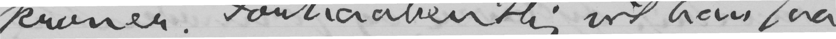kroner. Forhaabentlig vil han faa
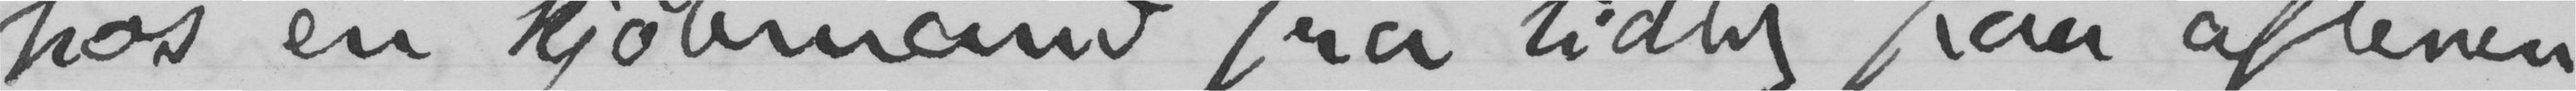hos en kjøbmand fra tidlig paa aftenen
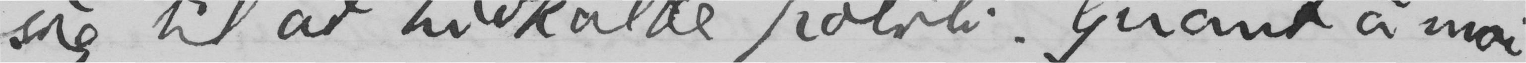sig til at hidkalde politi. Qùant à moi,
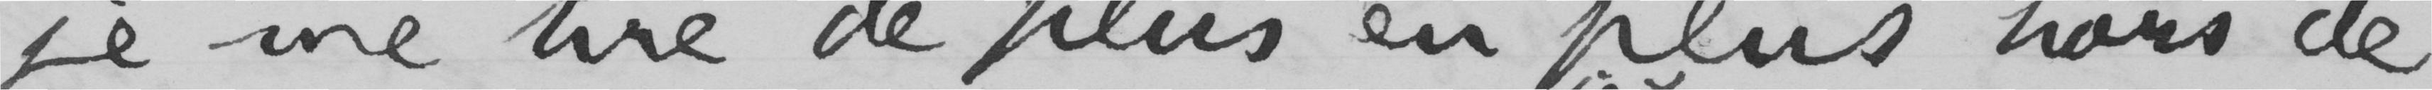jè me tire de plus en plus hors de
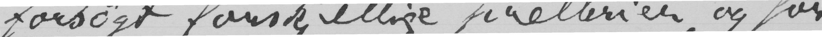forsøgt forskjellige prellerier, og for
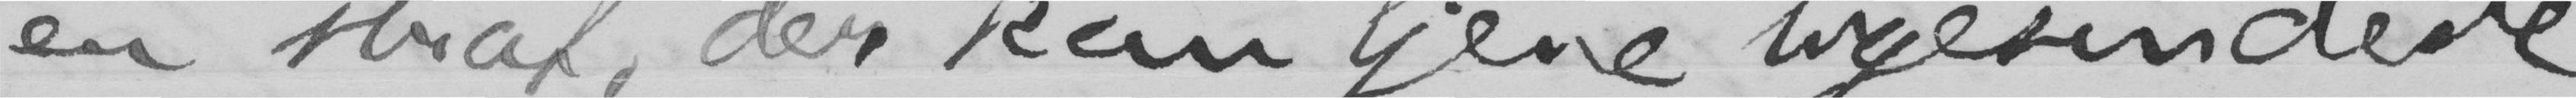en straf, der kan tjene ligesindede
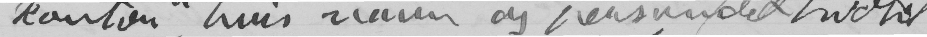kontor», hvis navn og person det hidtil
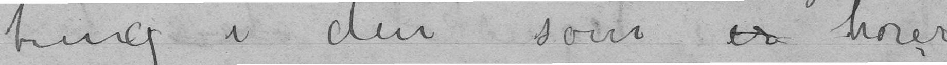ting i den som er hører
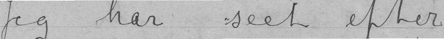Jeg har seet efter
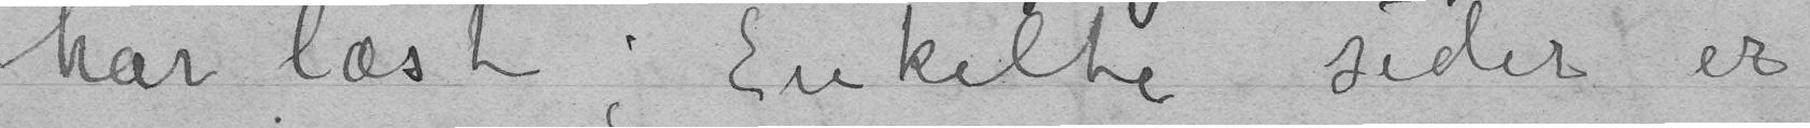har læst. Enkelte sider er
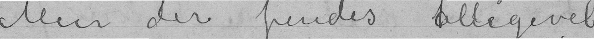Men der findes alligevel
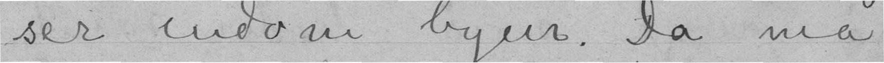ser indom byen. Du må
